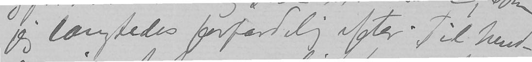jeg længtedes forfærdelig efter. Til hende
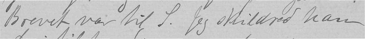Brevet var til S. Jeg skildred han
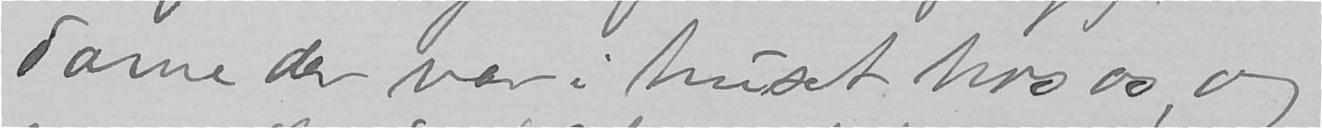dame der var i huset hos os, og
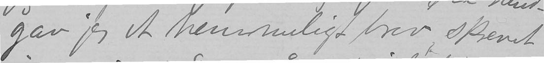gav jeg et hemmeligt brev, skrevet
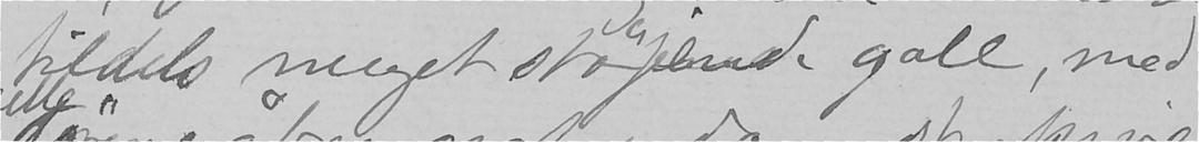tildels meget støjende gale, med
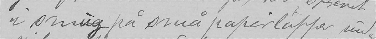i smug på små papirlapper under
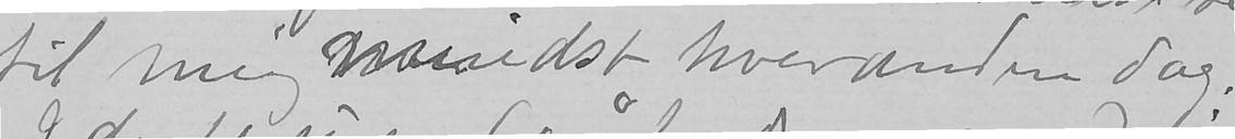til mig mindst hveranden dag.
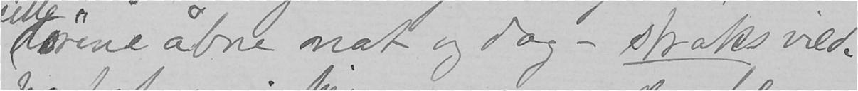dørene åbne nat og dag - straks vilde
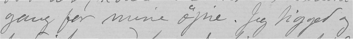gang for mine øjne. Jeg tigged og
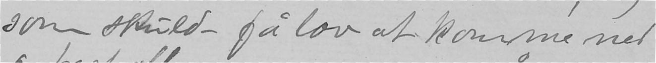som skulde få lov at komme ned
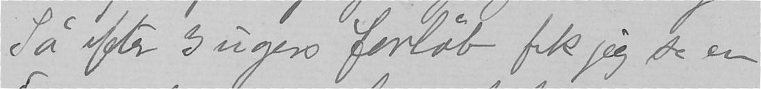Så efter 3 ugers forløb fik jeg se en
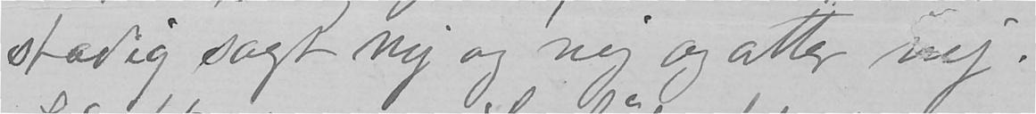stadig sagt nej og nej og atter nej.
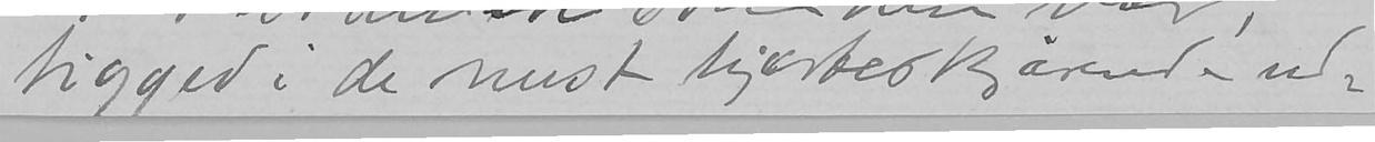tigged i de mest hjærteskjærende ud-
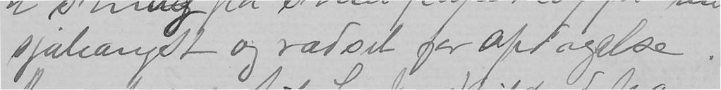sjæleangst og rædsel for opdagelse.
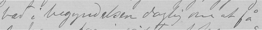bad i begyndelsen daglig om at få
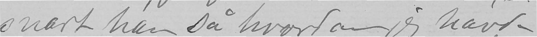snart han så hvordan jeg havde
